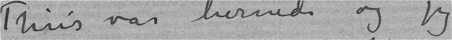Thiis var hernede og jeg
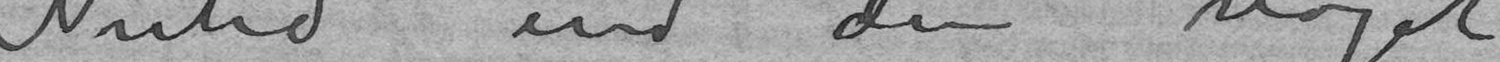Nutid end den noget
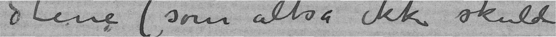Stene (som altså ikke skulde
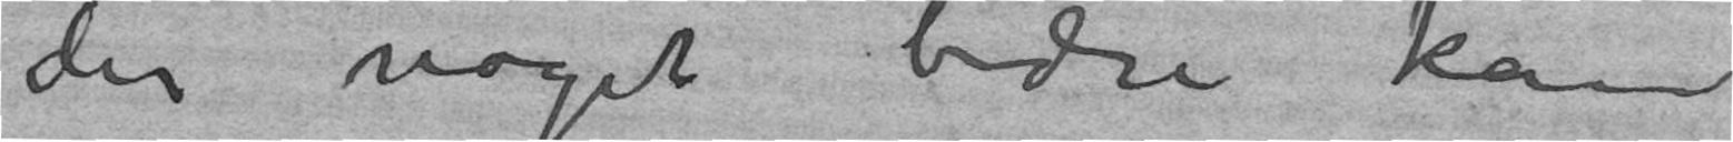der noget bedre kan
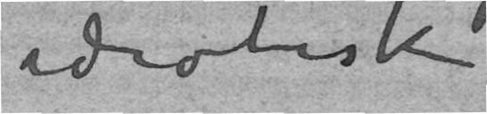idiotiske
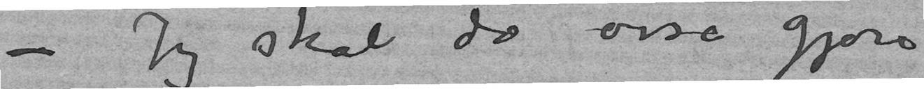– Jeg skal da ogsså gjøre
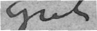gut
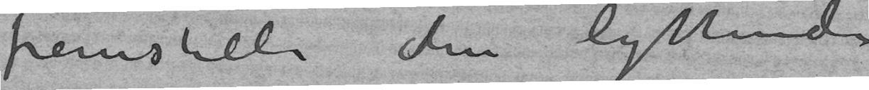fremstille den lyttende
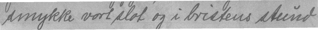smykke vort slot og i bristens stund
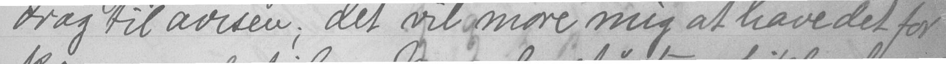drag til avisen; det vil more mig at have det for
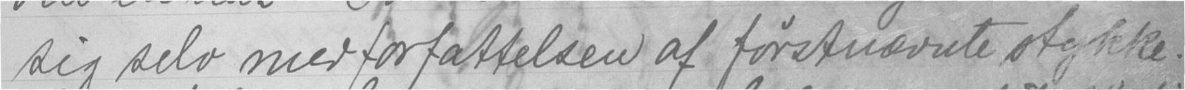sig selv med forfattelsen af forstnævnte stykke.
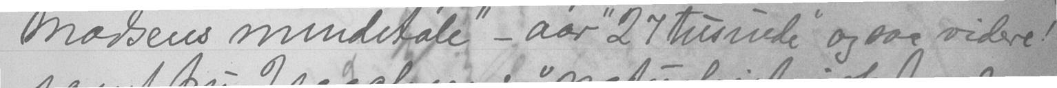Madsens mindetale" - aar "27 tusinde" og saa videre!
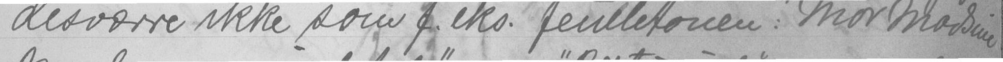desværre ikke som feks. feulletonen: "Mor Madsin
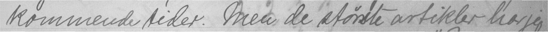kommende tider. Men de største artikler har jeg
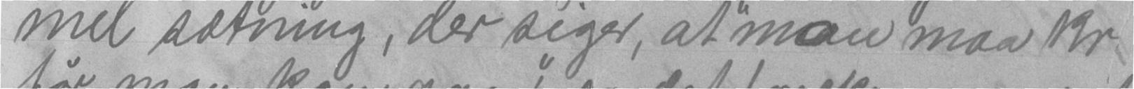mel sætning, der siger, at "man maa kr
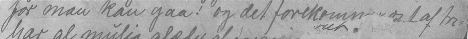før man kan gaa!" og det forekommer os af tr-
